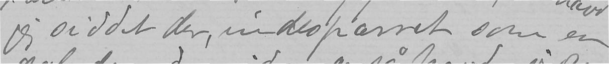jeg siddet der, indespærret som en
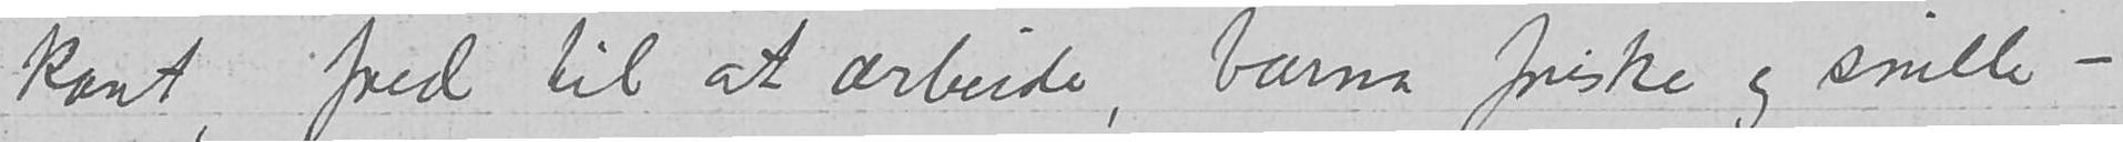kant, fred til at arbeide, barna friske og snille -
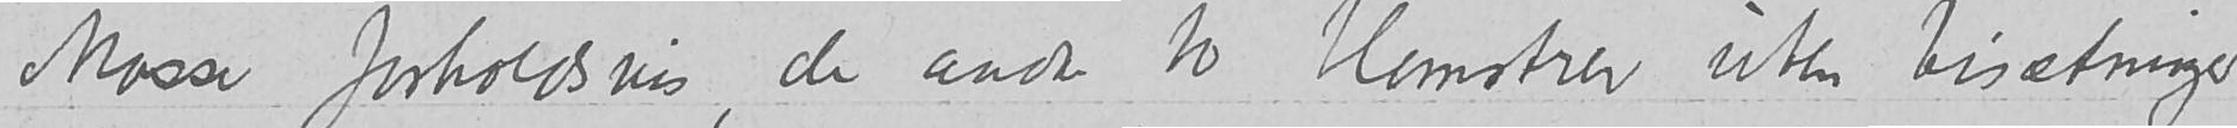Mosse forholdsvis, de andre to blomstrer uten bisætninger
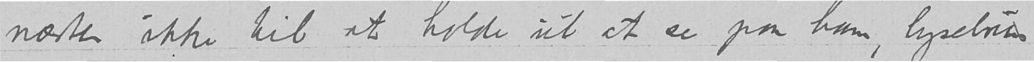næsten ikke til at holde ut at se paa ham, lysebrun
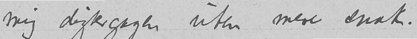mig digtergagen uten mere snak.
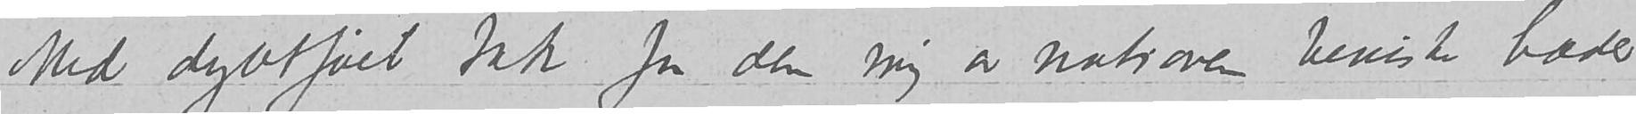Med dybtfølt tak for den mig av nationen beviste hæder
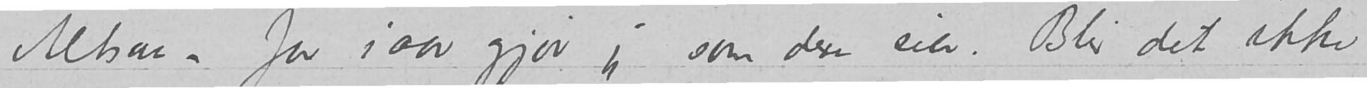Altsaa - for iaar gjør jeg som dere sier. Blir det ikke
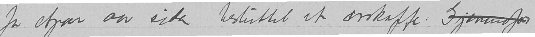for etpar aar siden besluttet at avskaffe. Gjennefor
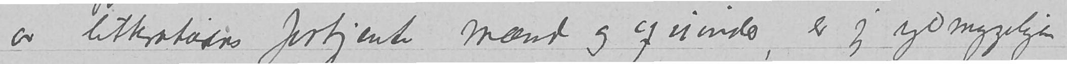av litteraturens fortjente mænd og qvinder, er jeg ydmygeligen
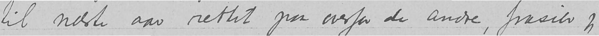til næste aar rettet paa overfor de andre, frasier jeg
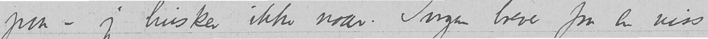paa - jeg husker ikke naar. Ingen breve fra en viss
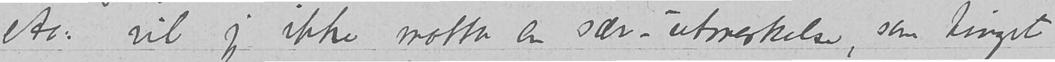etc. vil jeg ikke motta en sær-utmerkelse, som tinget
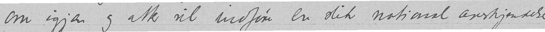om igjen og atter vil indføre en slik national anerkjendelse
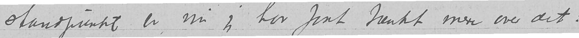standpunkt er nu jeg har faat tænkt mere over det:
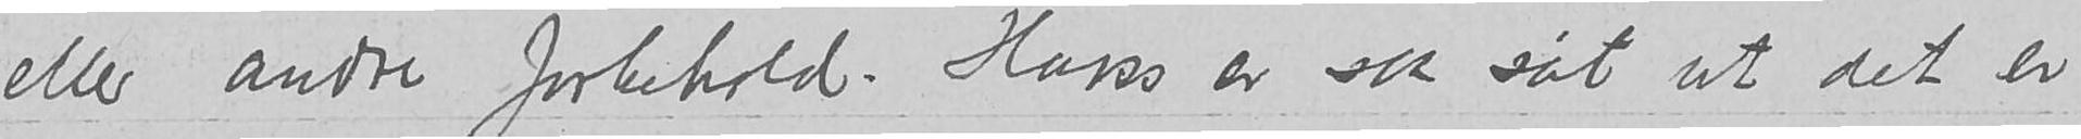eller andre forbehold. Hans er saa søt at det er
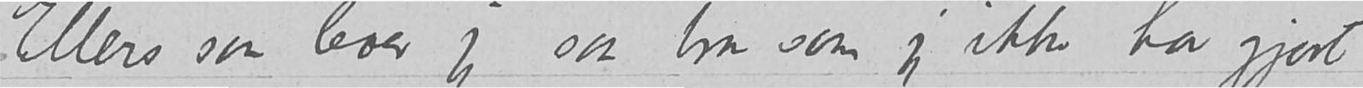Ellers saa lever jeg saa bra som jeg ikke har gjort
